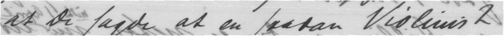at De sagde at en saadan Violinist
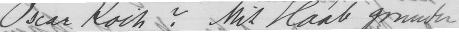Oscar Koch? Mit Haab grunder
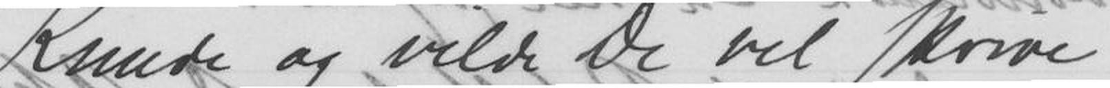Kunde og vilde De vel skrive
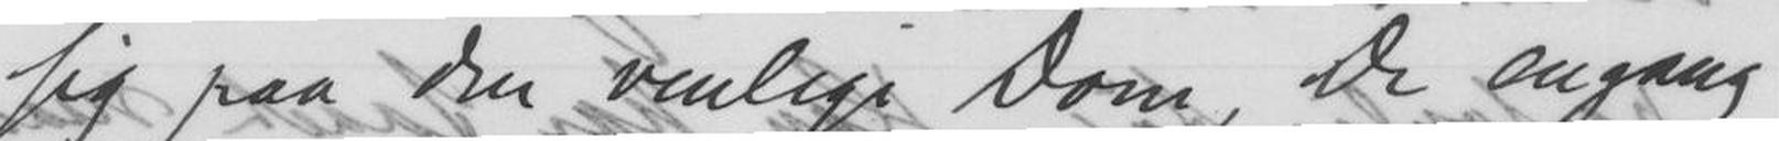sig paa den venlige Dom, De engang
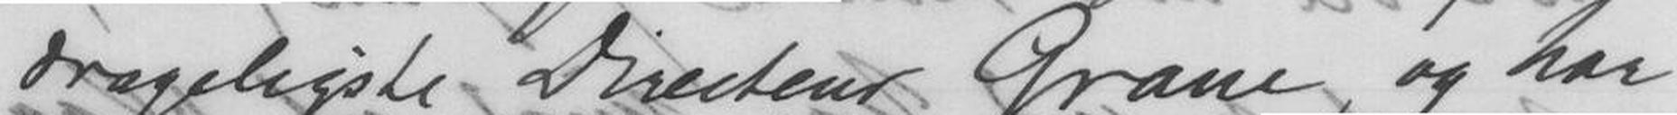drageligste Directeur Graue, og har
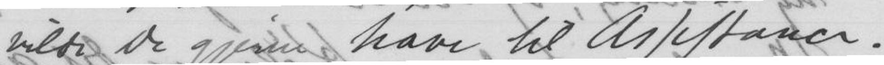vilde De gjerne have til Assistance
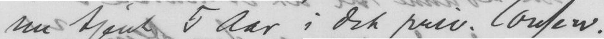nu tjent 5 Aar i det priv. Conserv.
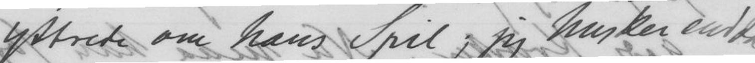yttrede om hans Spil; jeg husker endda
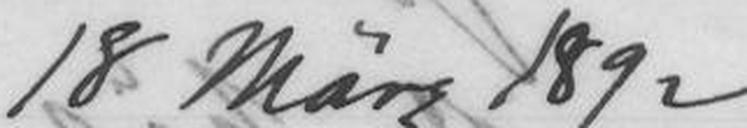18 März 1892
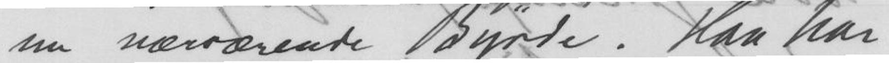nu nærværende Byrde. Han har
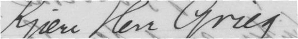Kjære Herr Grieg!
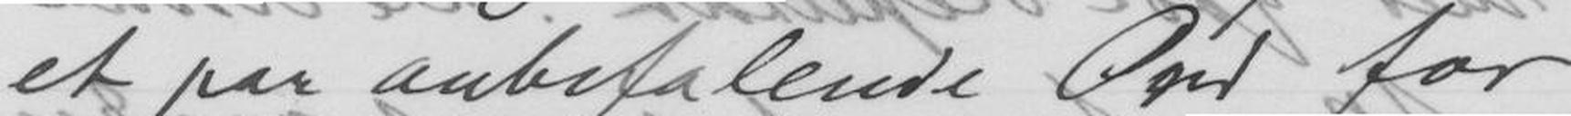et par anbefalende Ord for
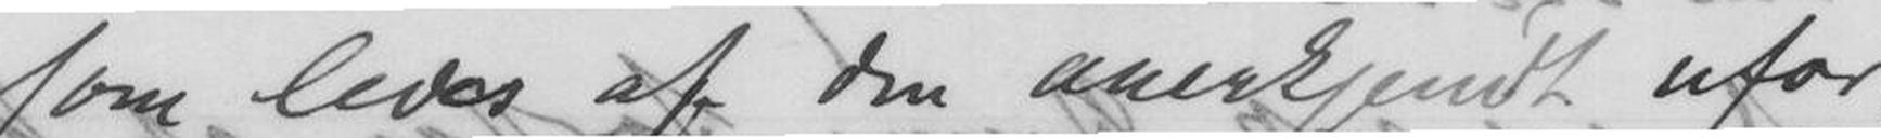som ledes af den anerkjendt ufor-
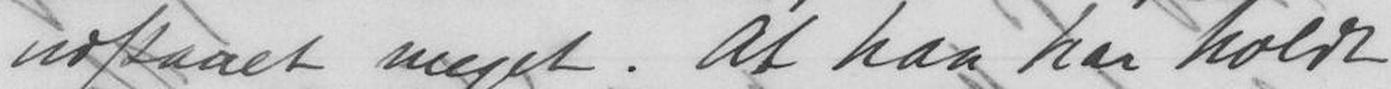udstaaet meget. At han har holdt
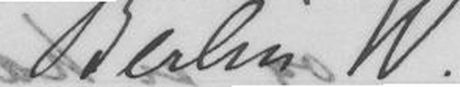Berlin W
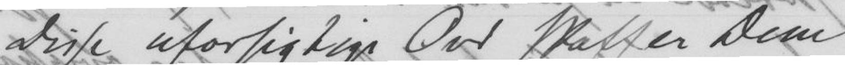Disse uforsigtige Ord skaffer Dem
